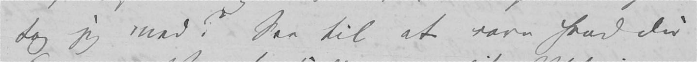tog jeg med? See til at vare hvad Du
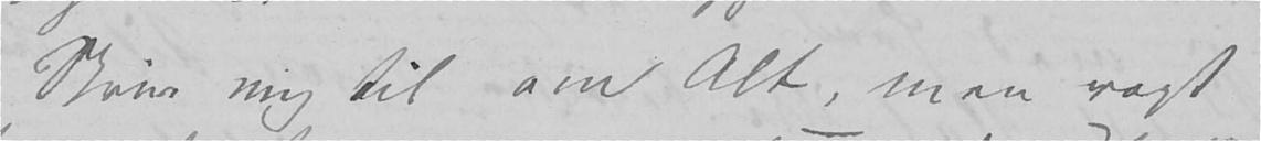Skriv mig til om Alt, men vogt
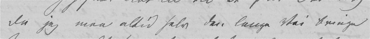da jeg maa altid selv den lange Vei bringe
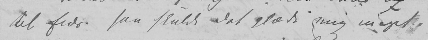til Eids. saa skulde det glæde mig meget.
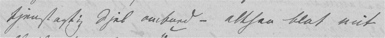til en tjenstagtig Sjel ombord – altsaa blot mit
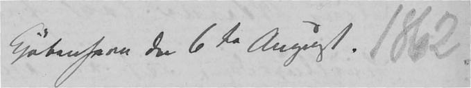Kjøbenhavn den 6te August.
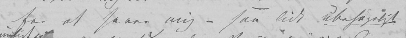for at saare mig – saa lidt ubehageligt
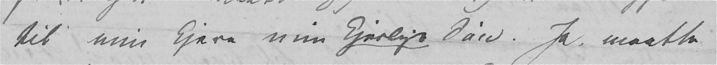til min kjere min kjerlige Søn. Ja, maatte
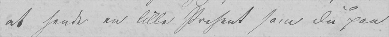at sende en lille Present som Du paa
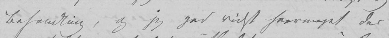Behandling, og jeg gad vidst hvormeget der
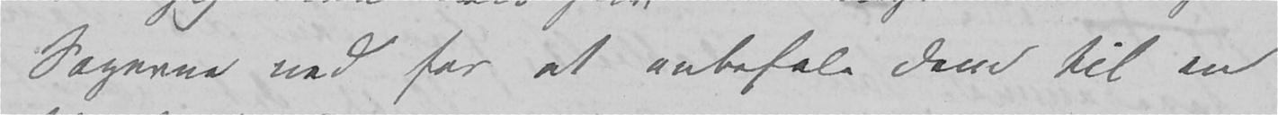Sagerne ned for at anbefale dem til en
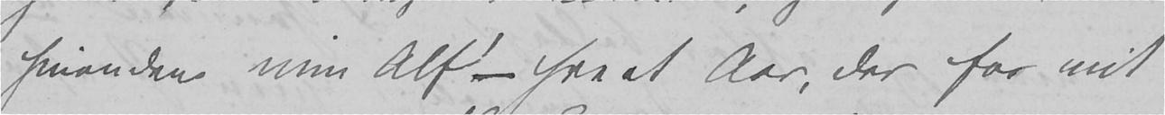hinanden min Alf – hvert Aar, der for mit
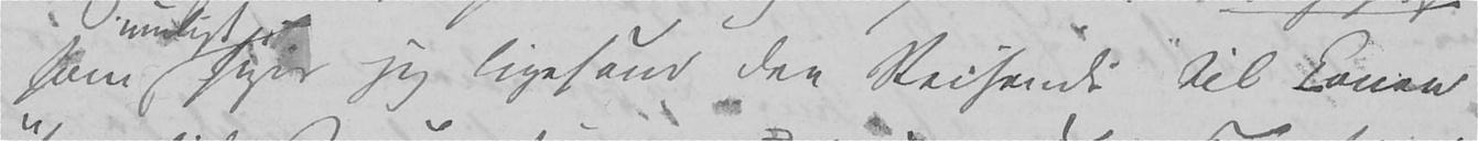som siger jeg ligesom den Reisende til Konen
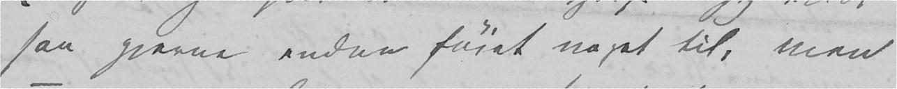saa gjerne endnu føiet noget til, men
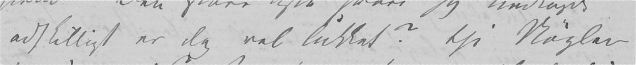adskilligt er dog vel lukket? thi Nøglen
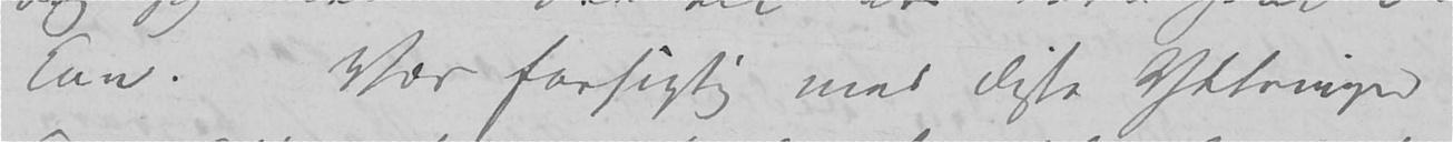kan. Vær forsigtig med disse Yttringer
